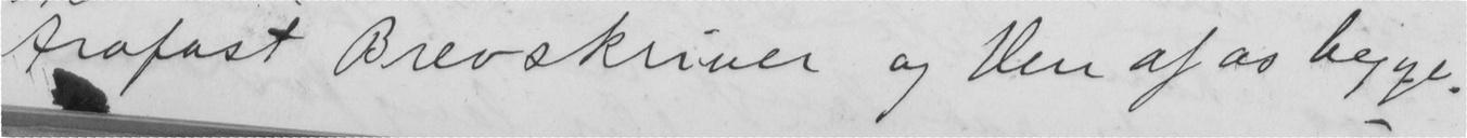trofast Brevskriver og Ven af os begge.
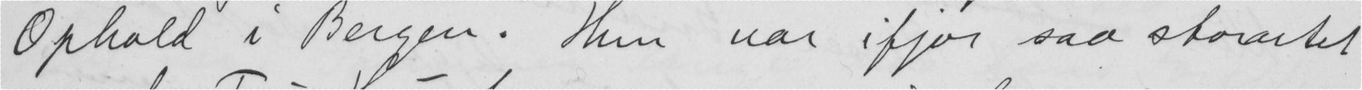Ophold i Bergen. Hun var ifjor saa storatet
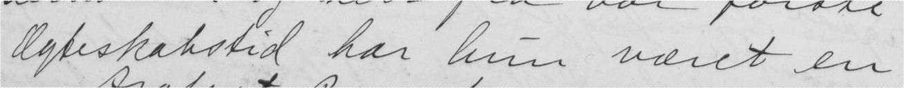Ægteskabstid har hun været en
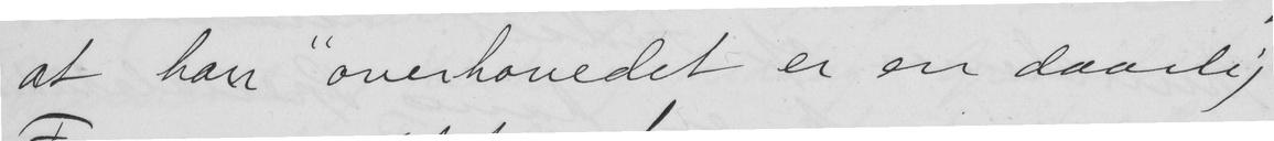at han "overhovedet er en daarlig
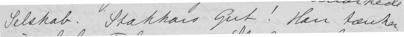Selskab. Stakkars Gut! Han tænker
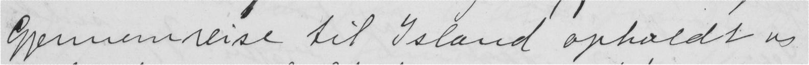gjennemreise til Island opholdt os
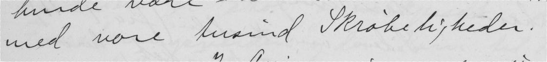med vore tusind Skrøbeligheder.
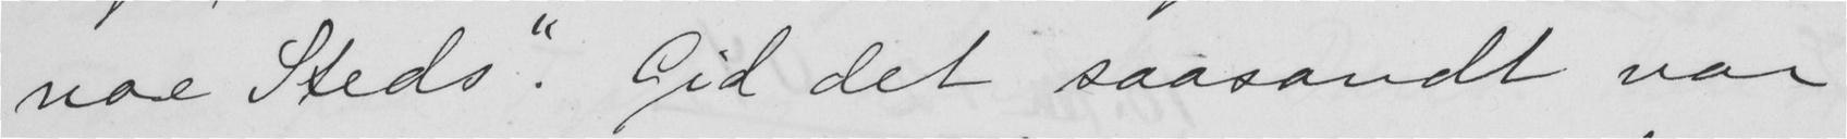noe Steds". Gid det saasandt var
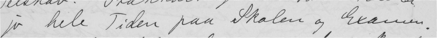jo hele Tiden paa Skolen og Examen
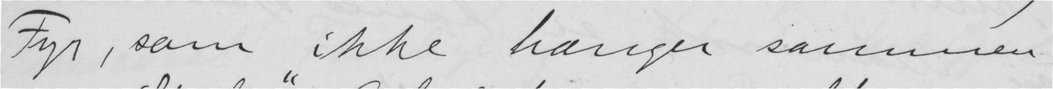Fyr, som ikke hænger sammen
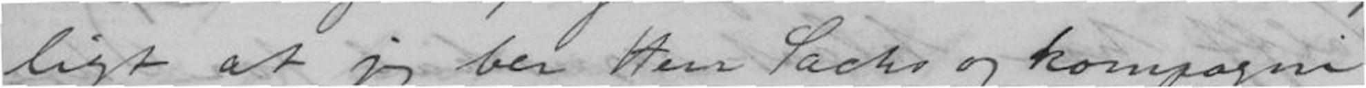ligt at jeg ber Herr Sachs og kompagni
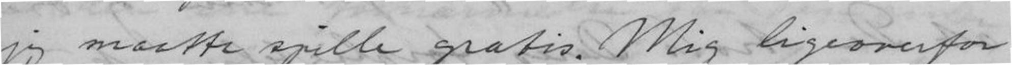jeg maatte spille gratis. Mig ligeoverfor
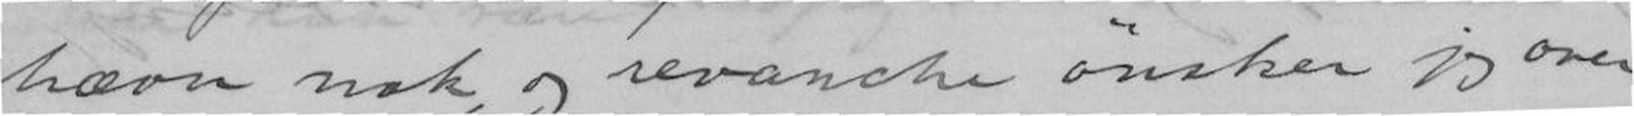hævn nok, og revanche ønsker jeg over
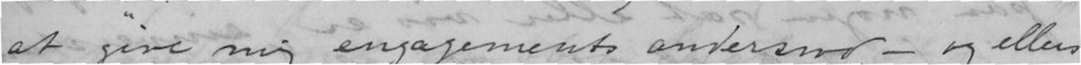at give mig engagements andersved, - og ellers
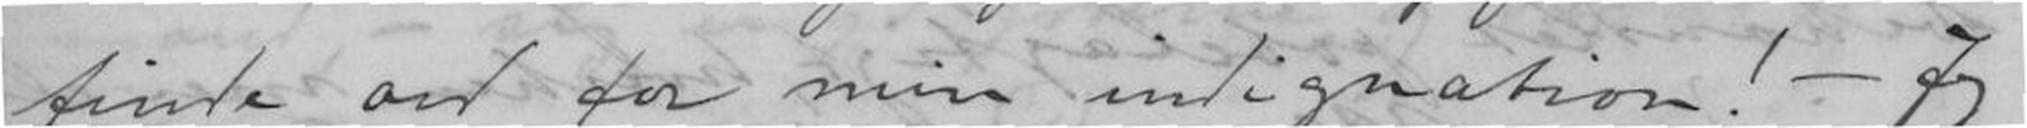finde ord for min indignation! - Jeg
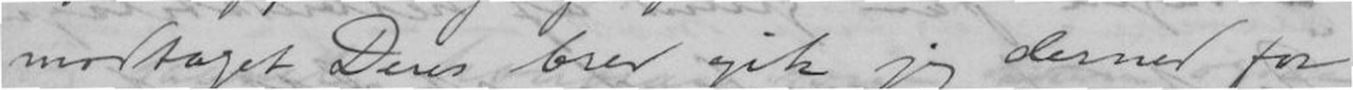modtaget Deres brev gik jeg derned for
